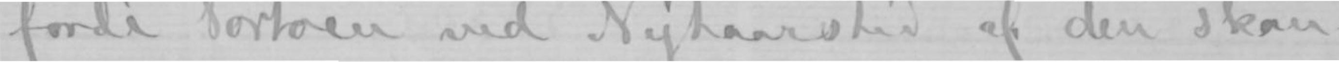fordi Portoen ved Nytaarstid af den skan-
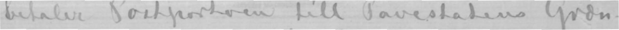betaler Postportoen till Pavestatens Græn-
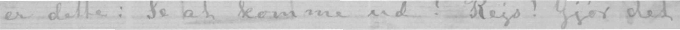er dette: Se at komme ud! Rejs! Gjør det
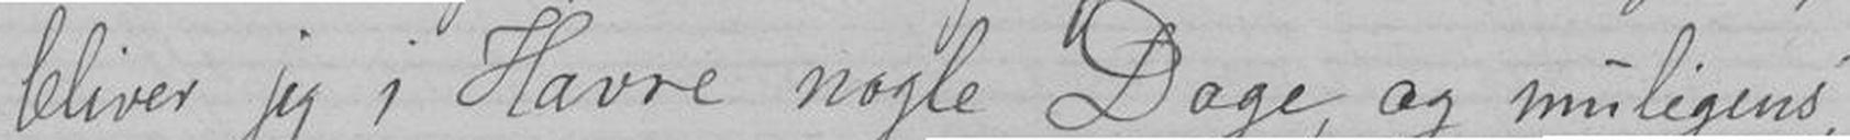bliver jeg i Havre nogle Dage, og muligens,
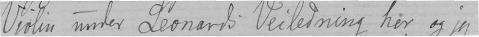Violin under Leonards Veiledning her, og jeg
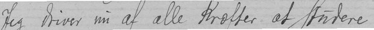Jeg driver nu af alle Kræfter at studere
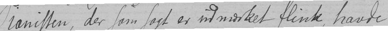Pianisten, der som sagt er udmærket flink, havde
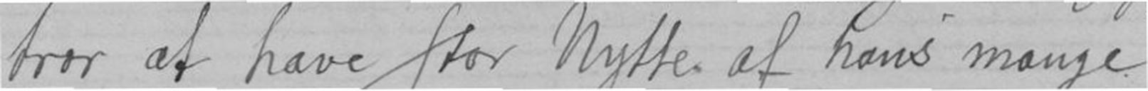tror at have stor Nytte af hans mange
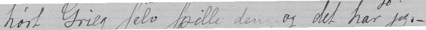hørt Grieg selv spille den, og det har jeg. -
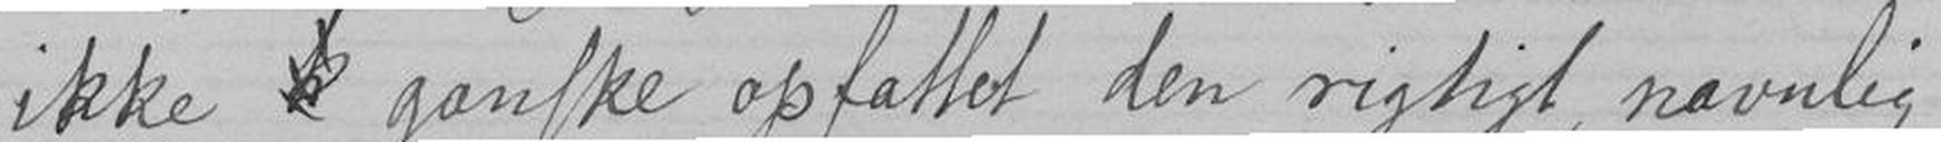ikke k ganske opfattet den rigtigt, navnlig
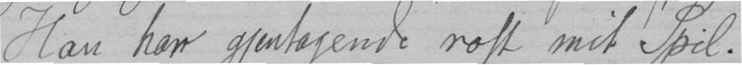Han har gjentagende rost mit Spil.
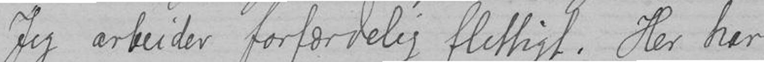Jeg arbeider forfærdelig flittigt. Her har
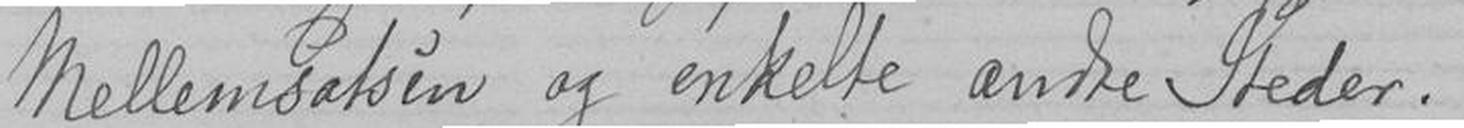Mellemsatsen og enkelte andre Steder.
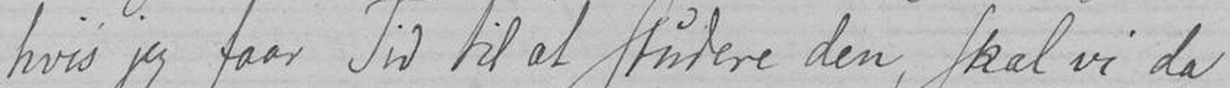hvis jeg faar Tid til at studere den, skal vi da
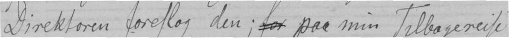Direktoren foreslog den; paa min Tilbagereise
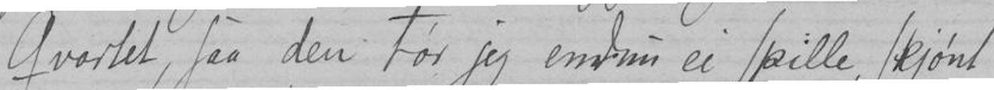Qvartet, saa den tør jeg endnu ei spille, skjønt
